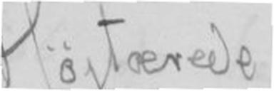Højtærede
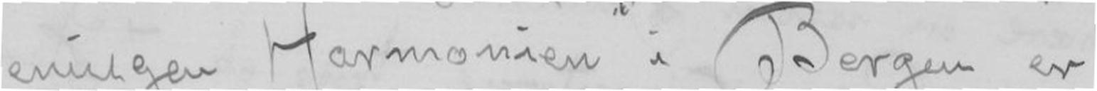eningen "Harmonien" i Bergen er
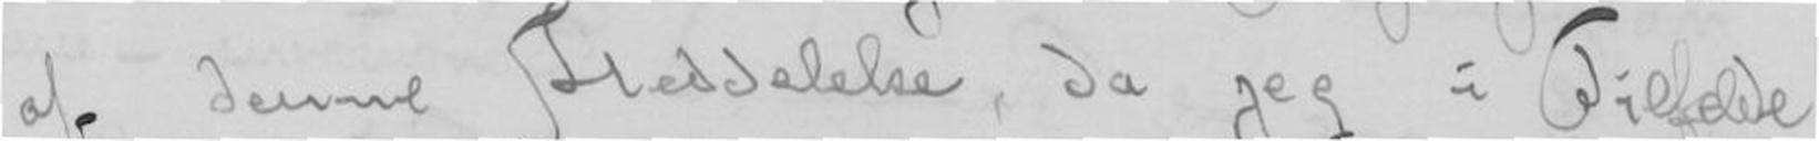af denne Meddelelse, da jeg i Tilfelde
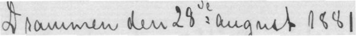Drammen den 28de August 1881,
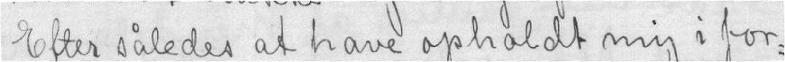Efter således at have opholdt mig i for-
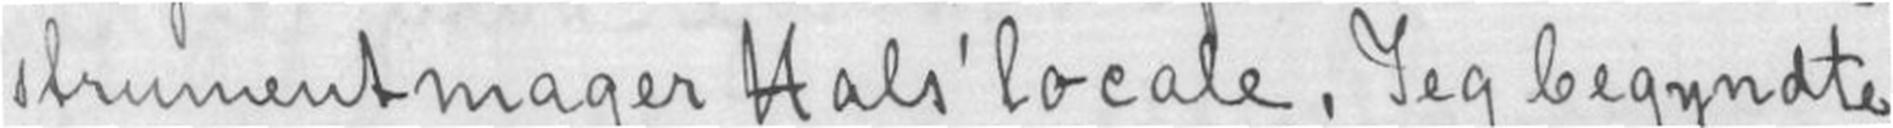strumentmager Hals'locale. Jeg begyndte
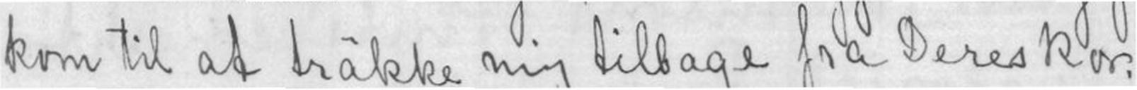kom til at träkke mig tilbage fra Deres kor.
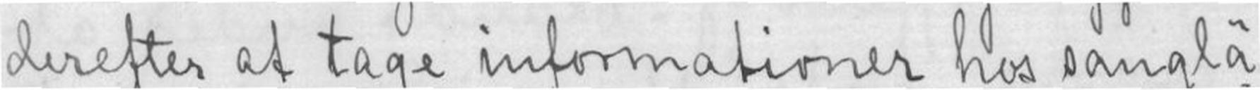derefter at tage informationer hos sanglä-
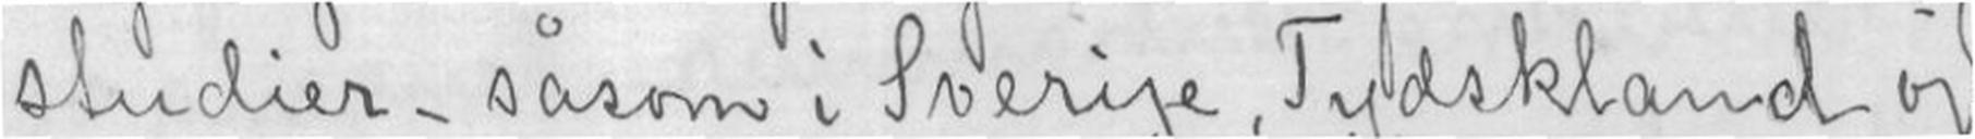studier - såsom i Sverige, Tydskland og
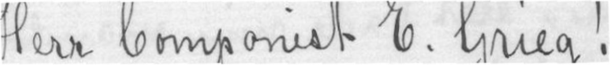Herr Componist E. Grieg!
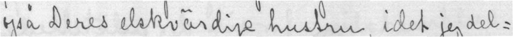også Deres elskvärdige hustru, idet jeg del-
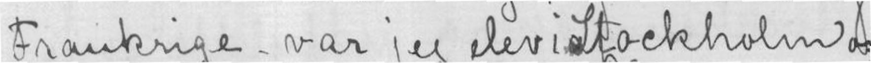Frankrike, var jeg elev i Stockholm og
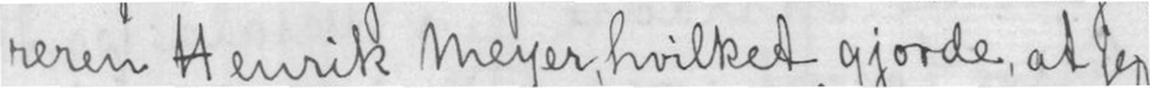reren Henrik Meyer, hvilket gjorde, at jeg
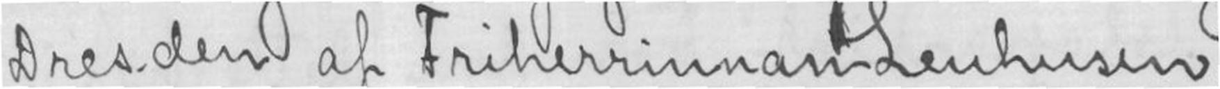Dresden af Friherrinnan Lenhusen,
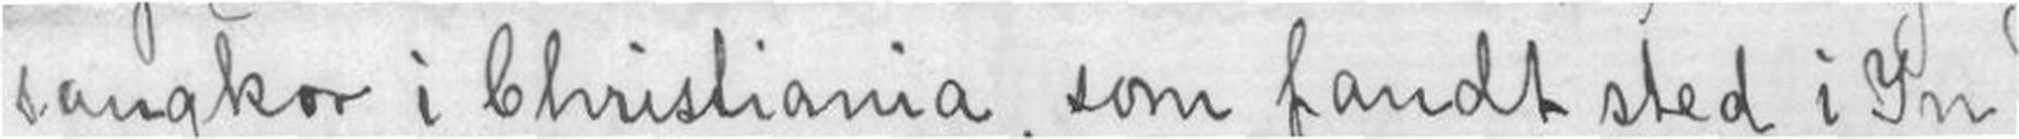sangkor i Christiania, som fandt sted i In-
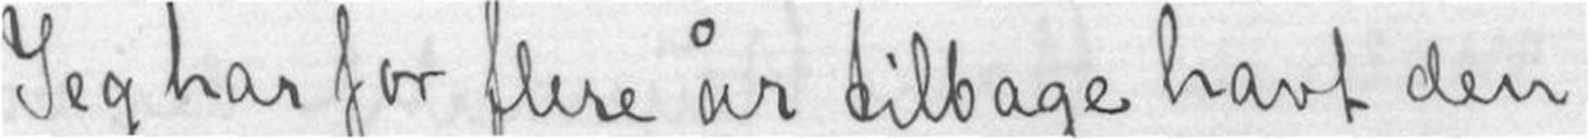Jeg har for flere år tilbage havt den
